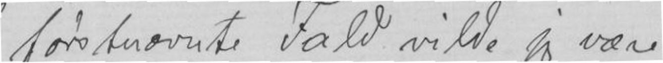I førstnævnte Fald vilde jeg være
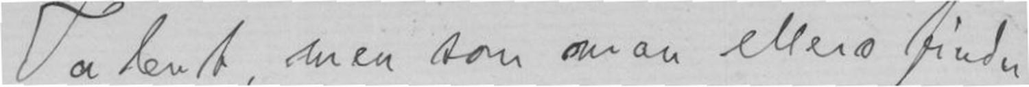Talent, men som man ellers finder
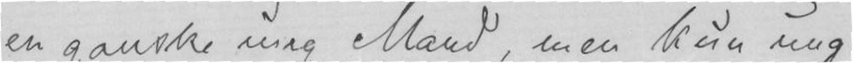en ganske ung Mand, men kun ung
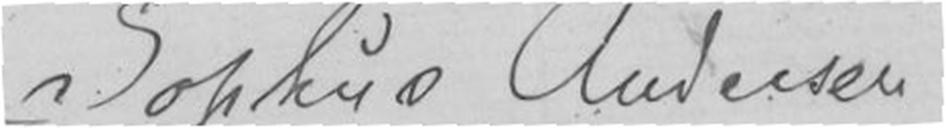Sophus Andersen
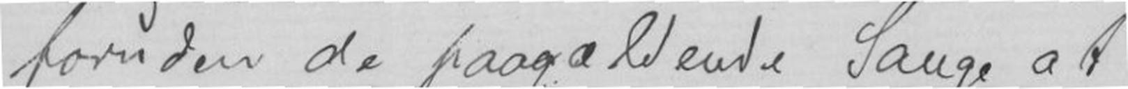foruden de paagældende Sange at
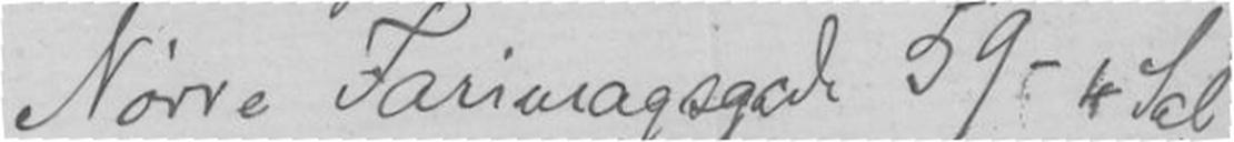Nørre Farimagsgade 59-4 Sal
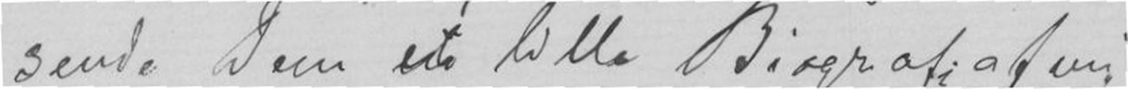sende Dem et lille Biografi af mig
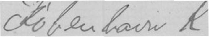København K.
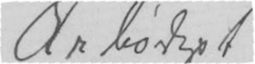Ærbødigst
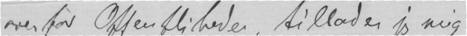overfor Offentligheden, tillader jeg mig
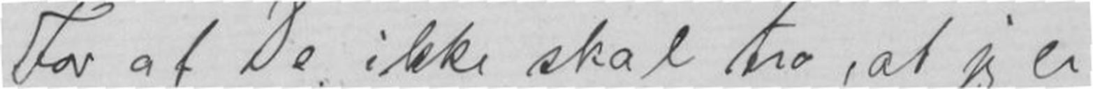For at De ikke skal tro, at jeg er
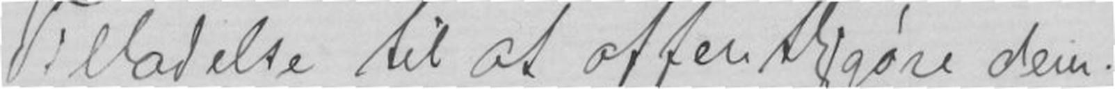Tilladelse til at offentliggøre dem.
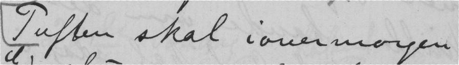Tuften skal iovermorgen
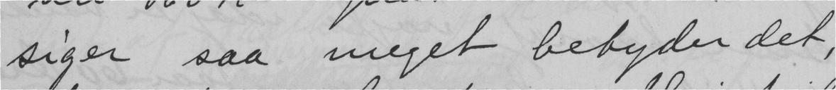siger saa meget bebyder det,
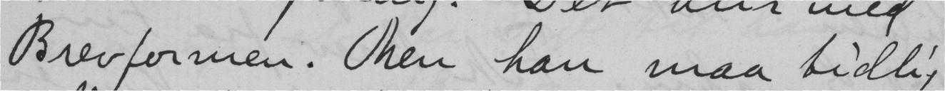Brevformen. Men han maa tidlig
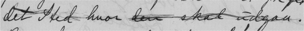det Sted hvor den skal udgaa.
